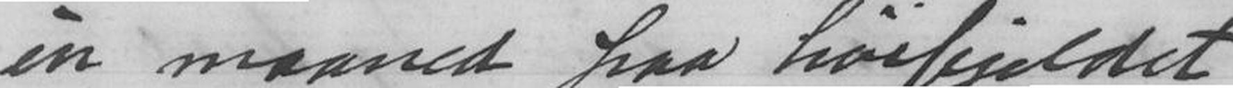èn maaned paa høifjeldet
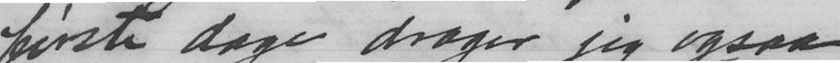første dage drager jeg ogsaa
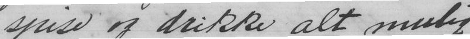spise og drikke alt mulig
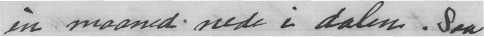èn maaned nede i dalen. Saa
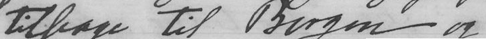tilbage til Borgen og
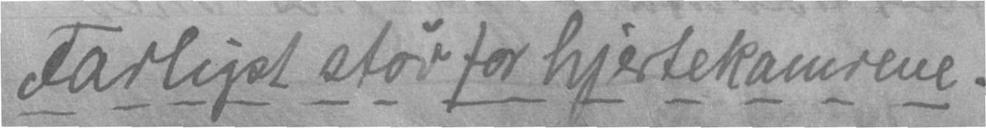Farligst støv for hjertekamrene.
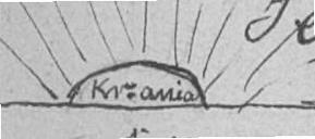Kr. ania
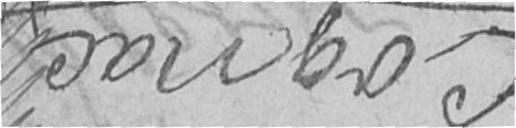Cognac
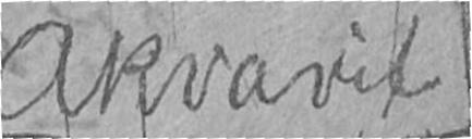Akvavit
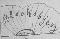Blocksbjerg
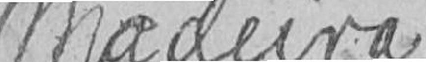Madeira
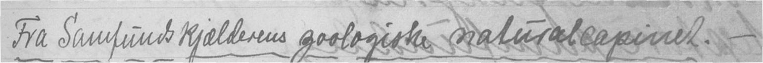Fra Samfundskjælderens geologiske naturalcapinet. -
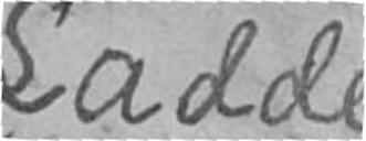Saddevin
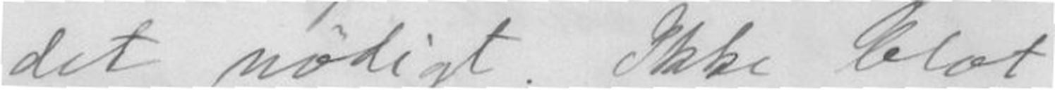det nødigt. Ikke blot
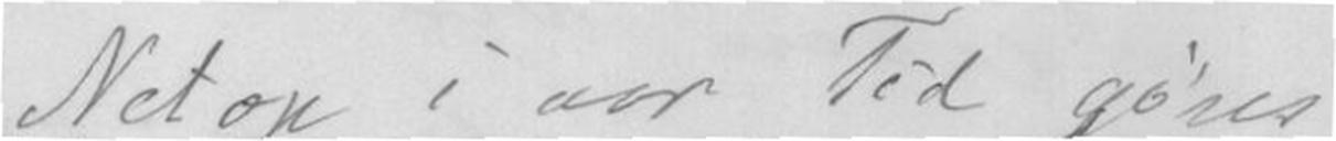Netop i vor Tid gøres
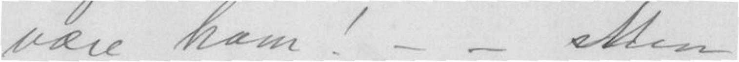være ham! - - Men
